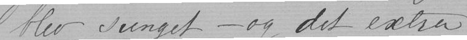blev sunget - og det extra
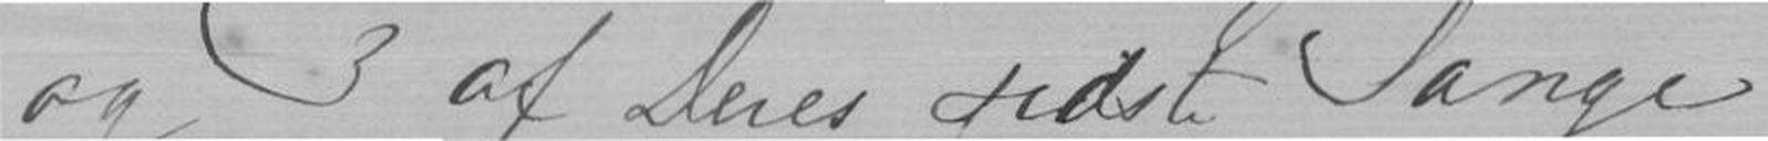og 3 af Deres sidste Sange
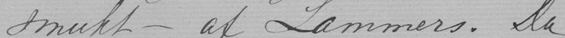smukt - af Lammers. Da
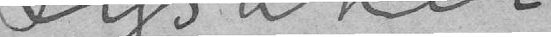Lysaker
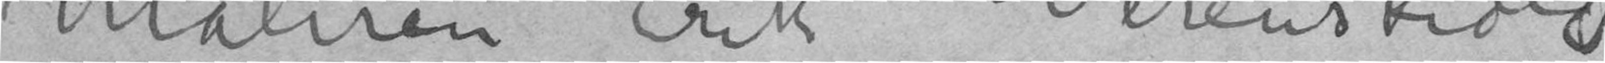Maleren Erik Werenskiold
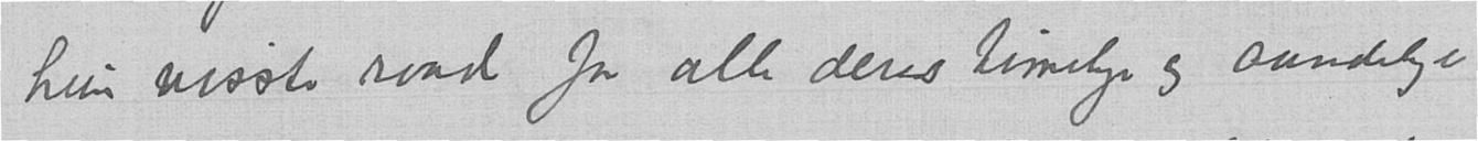hun visste raad for alle deres timelige og aandelige
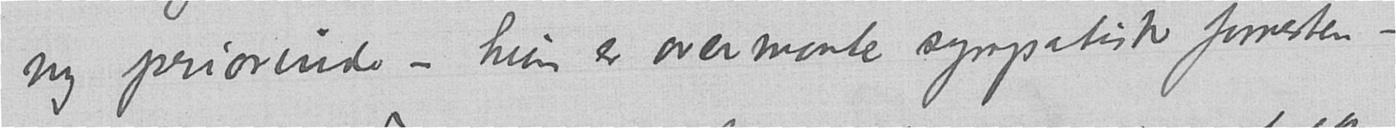ny priorinde – hun er overmaate sympatisk forresten –
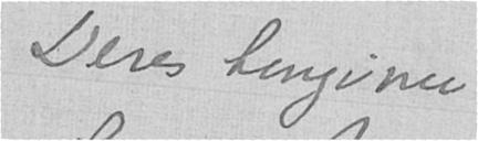Deres hengivne
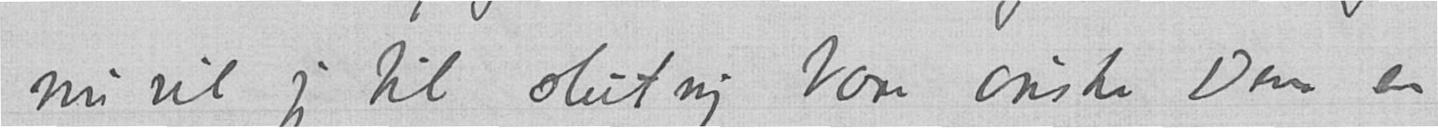nu vil jeg til slutnig bare ønske Dem en
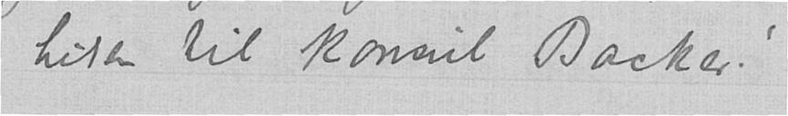hilsen til konsul Backer!
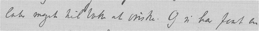later meget tilbake at ønske. Og vi har faat en
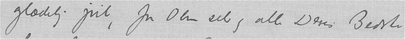glædelig jul, for Dem selv og alle Deres. Bedste
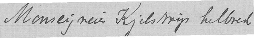Monseigneur Kjelstrups helbred
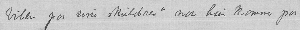bilen paa sine skuldrer" naar hun kommer paa
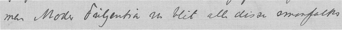men Moder Fulgentia var blit alle disse smaafolks
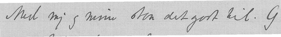Med mig og mine staar det godt til. Og
